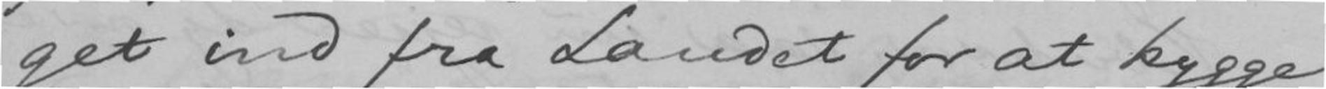get ind fra Landet for at hygge
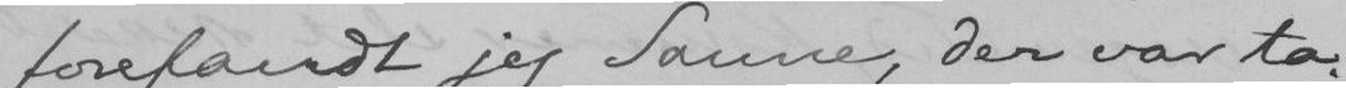forefandt jeg Sanne, der var ta-
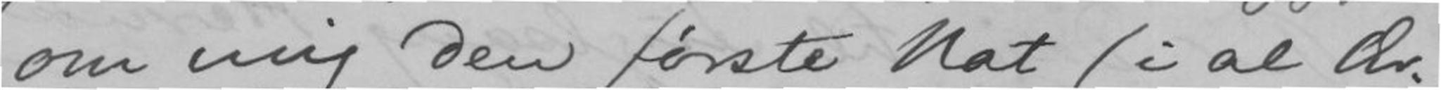om mig den første Nat (i al Ær-
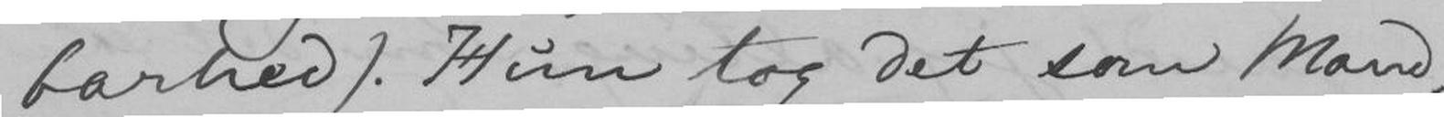barhed). Hun tog det som Mand,
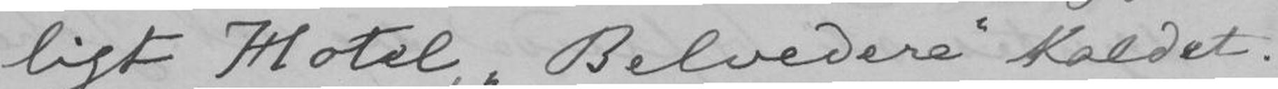ligt Hotel, Belvedere Kaldet.
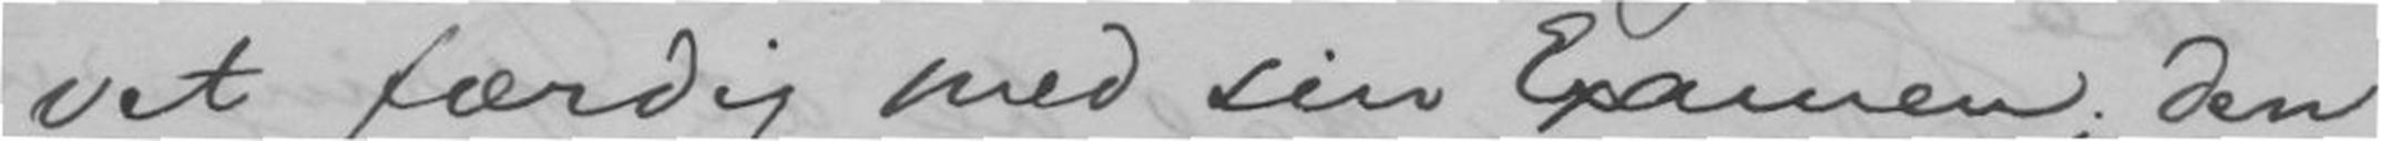vet færdig med sin Examen; den
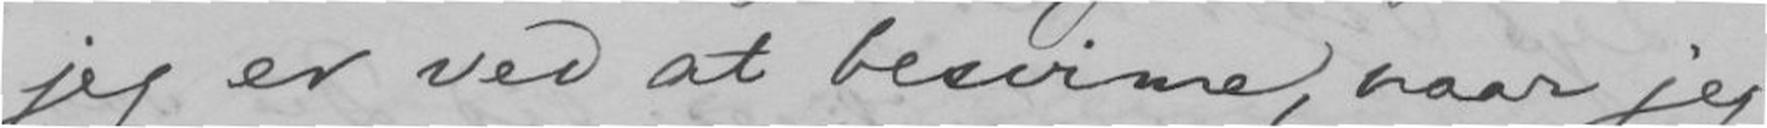jeg er ved at besvime, naar jeg
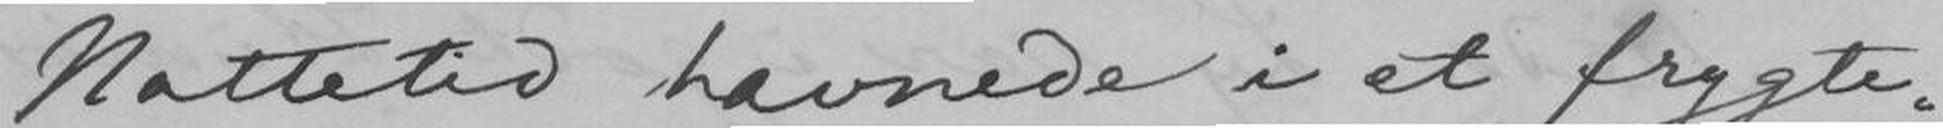Nattetid havnede i et frygte-
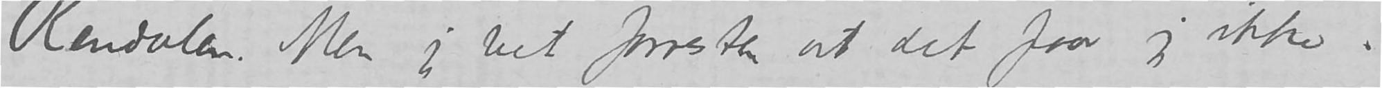Rendalen. Men jeg vet forresten at det faar jeg ikke.
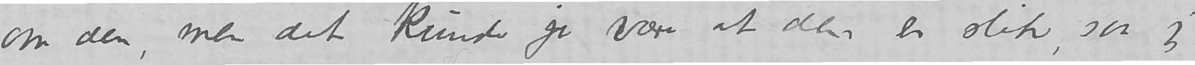om den, men det kunde jo være at den er slik, saa jeg
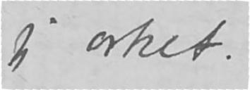jeg orket
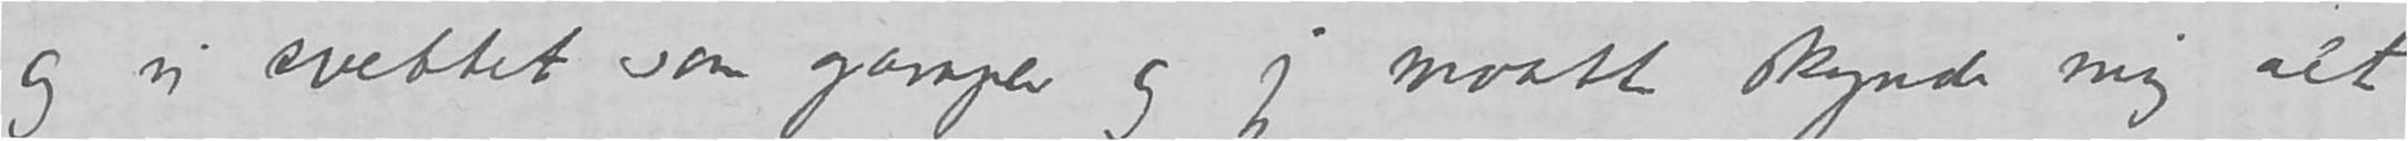og vi svettet som gamper og jeg maatte skynde mig alt.
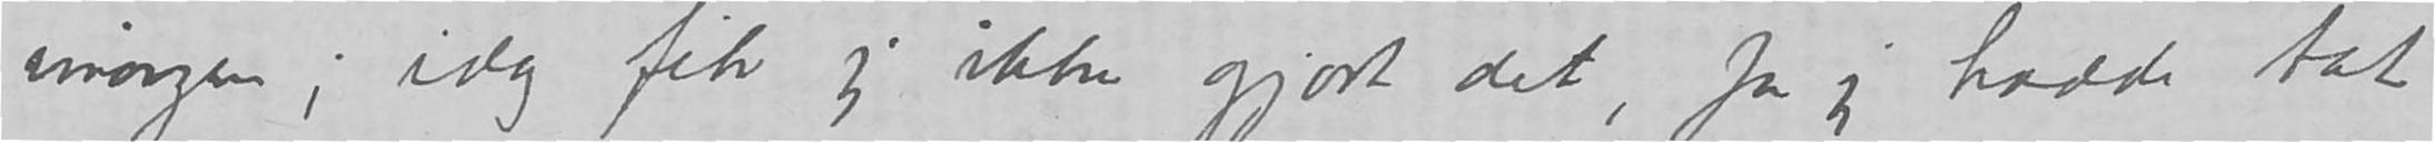imorgen; idag fik jeg ikke gjort det, for jeg hadde tat
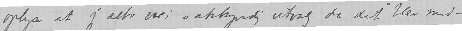oplyse at jeg selv var i sakkyndig utvalg da det blev med
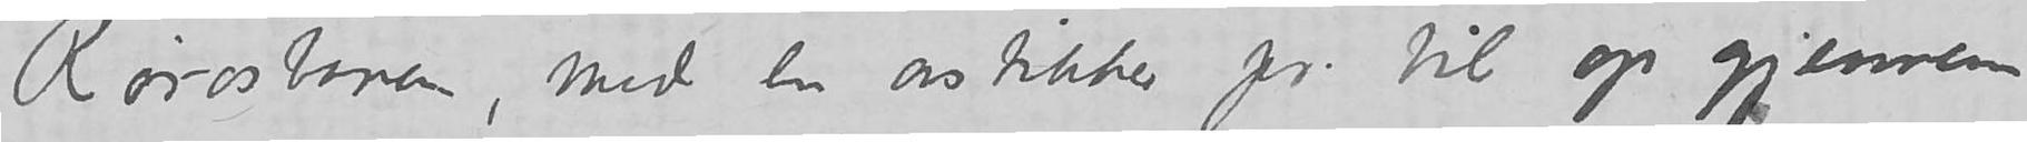Rørosbanen, med en avstikker fra til op gjennem
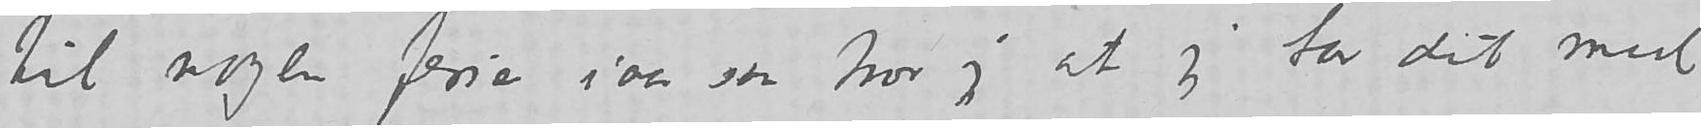til noen ferie iaar saa tror jeg at jeg tar dit med
